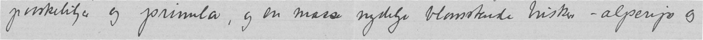paaskeliljer og primula, og en masse nydelige blomstrende busker – alperips og
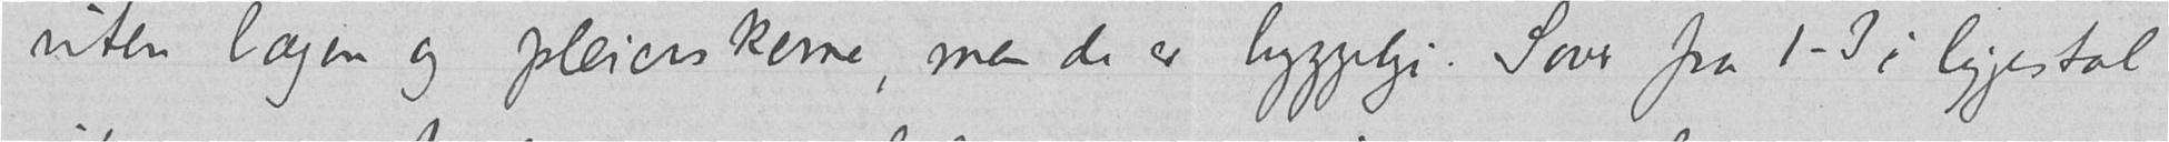uten lægen og pleierskerne, men de er hyggelige. Sover fra 1-3 i liggestol
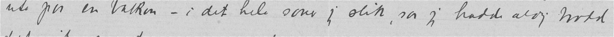ute paa en balkon – i det hele sover jeg slik, saa jeg hadde aldrig trodd
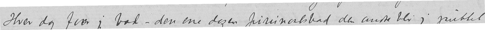Hver dag faar jeg bad – den ene dagen furunaalsbad, den andre blir jeg puttet
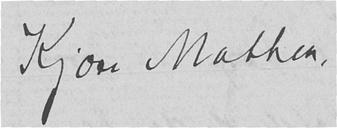Kjære Mathea,
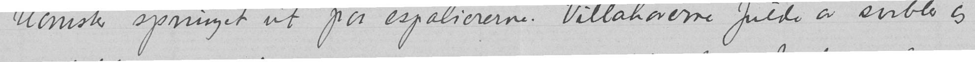blomster sprunget ut paa espaliererne. Villahaverne fulde av svibler og
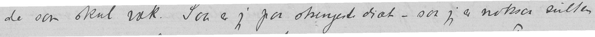de som skal væk. Saa er jeg paa strengeste diæt – saa jeg er noksaa sulten
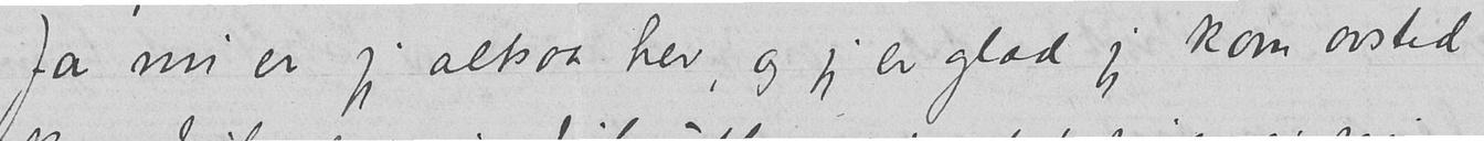Ja nu er jeg altsaa her, og jeg er glad jeg kom avsted
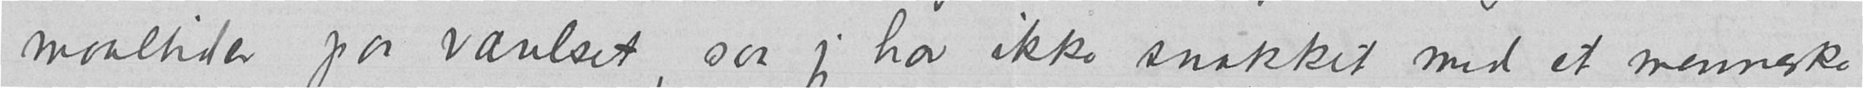maaltider paa værelset saa jeg har ikke snakket med et menneske
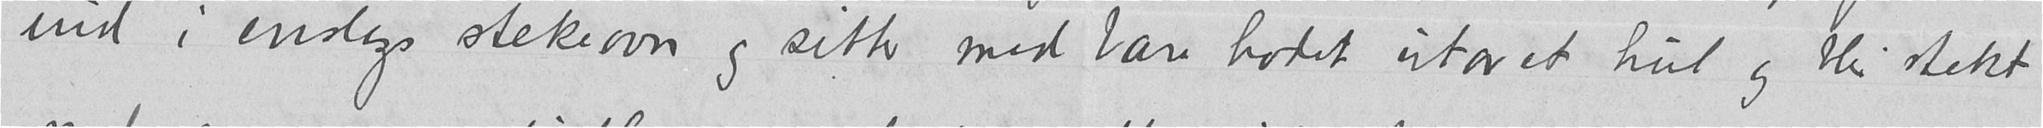ind i enslags stekeovn og sitter med bare hodet utav et hul og blir stekt
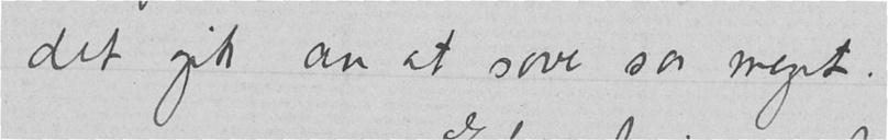det gik an at sove saa meget.
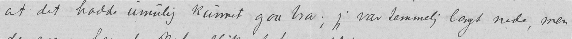at det hadde umulig kunnet gaa bra; jeg var temmelig langt nede, men
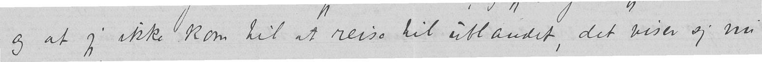og at jeg ikke kom til at reise til utlandet, det viser sig nu
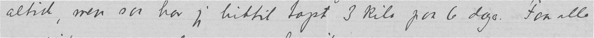altid, men saa har jeg hittil tapt 3 kilo paa 6 dager. Faar alle
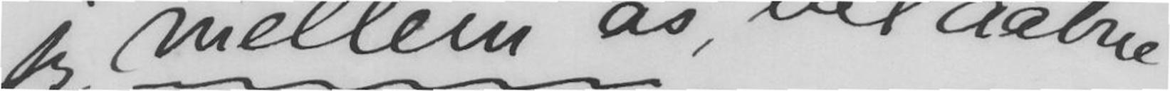jeg, mellem os, vil aabne
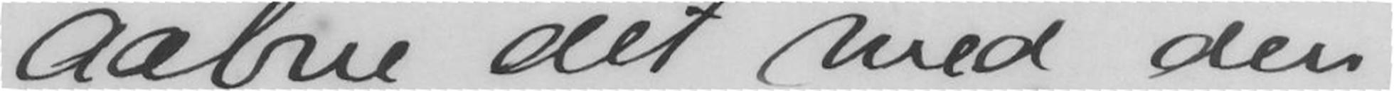Aabne det med den
